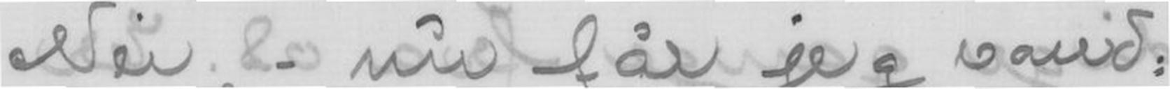Nei, - nu får jeg vand-
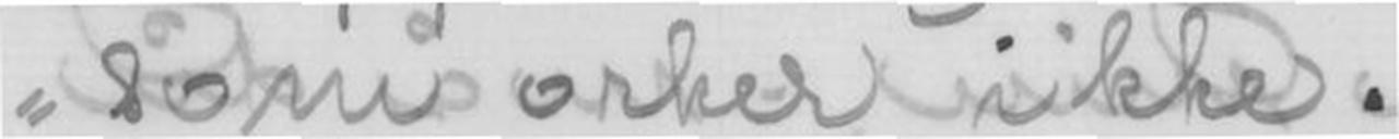som orker ikke.
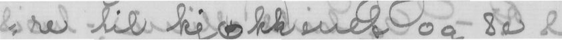re til kjøkkenet og se
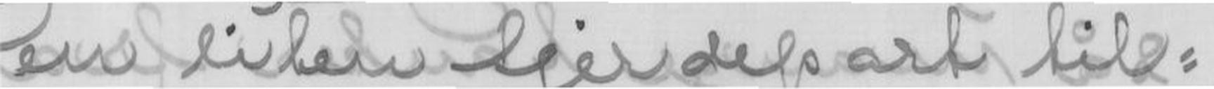en liten fjerdepart til-
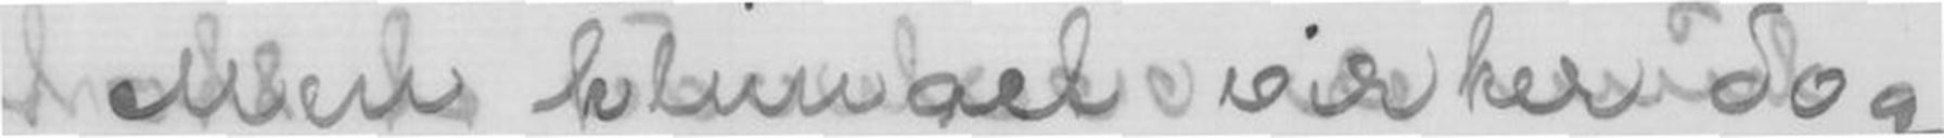Men klimaet virker dog
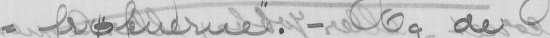= frøkerne. - Og de
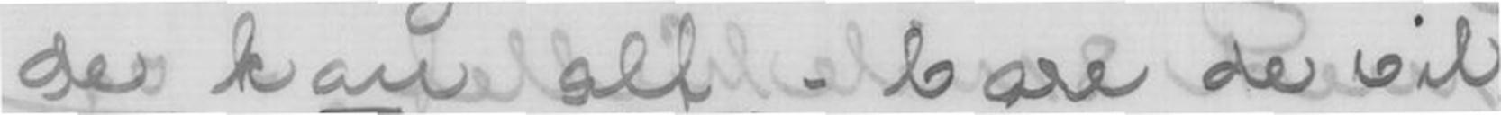de kan alt, - bare de vil,
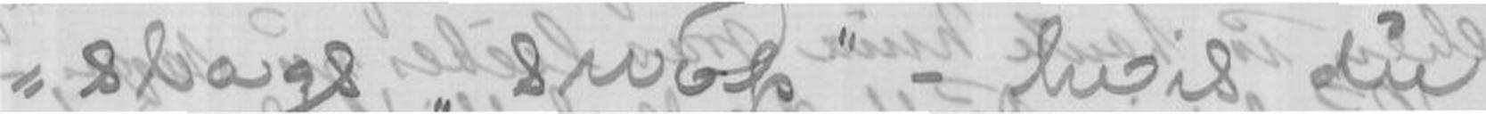slags snop - hvis du
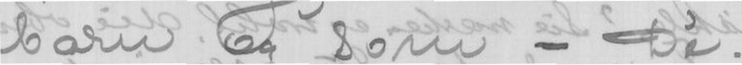barn og som - Dé.
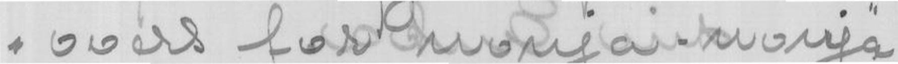overs for nonja-nonja
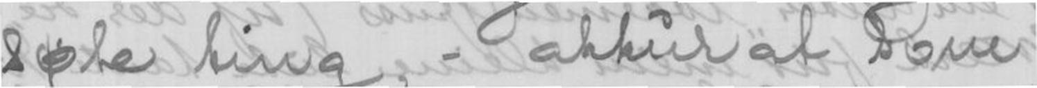søte ting, - akkurat som
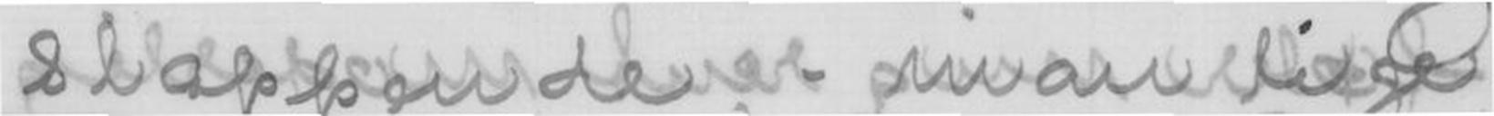slappende, - man lige-
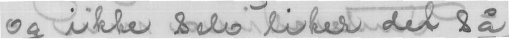og ikke selv liker det så
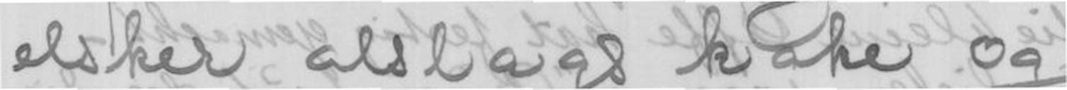elsker alslags kake og
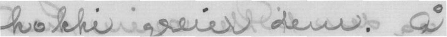kokke greier dem. Å -
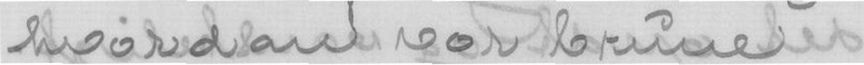hvordan vor brune
